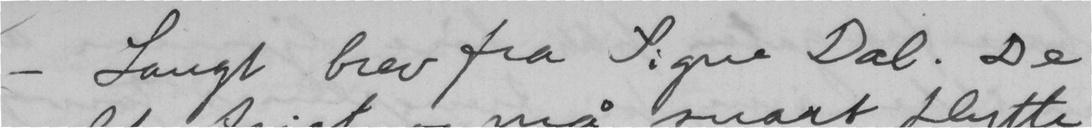- Langt brev fra Signe Dal. De
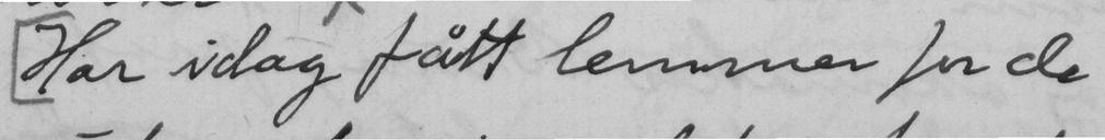Har idag fått lemmer for de
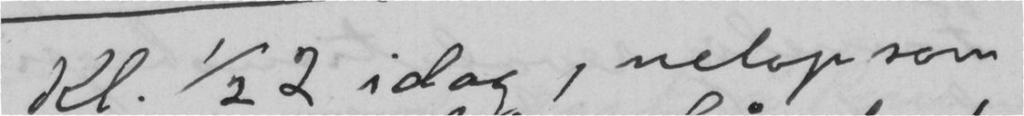Kl. ½ 2 idag, netop som
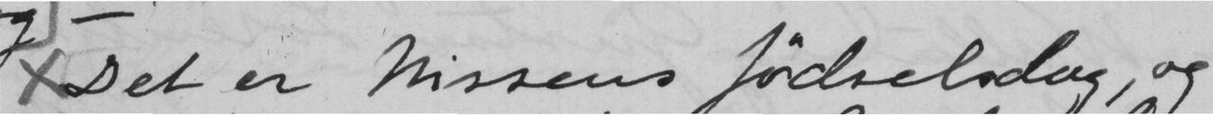Det er Nissens fødselsdag, og
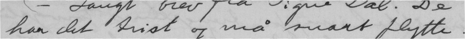har det trist og må snart flytte.
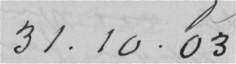31.10.03
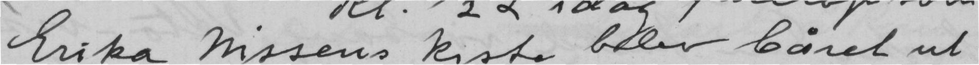Erika Nissens kiste blev båret ut
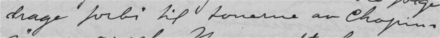drage forbi til tonerne av Chopnins
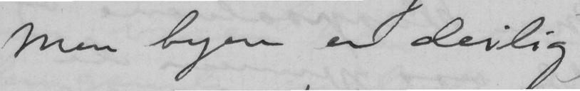Men byen er deilig
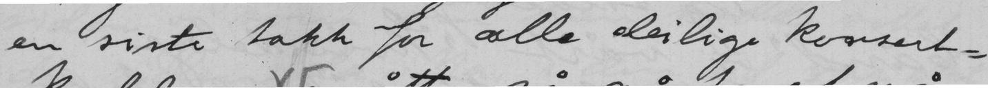en siste takk for alle deilige konsert-
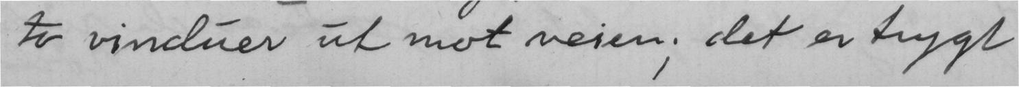to vinduer ut mot veien; det er trygt
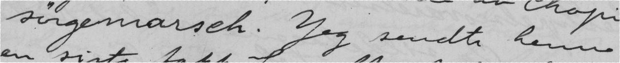sørgemarsch. Jeg sendte henne
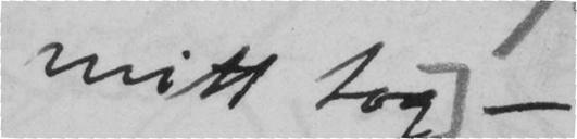mitt tog —
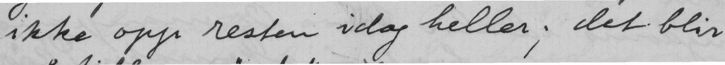ikke opp resten idag heller; det blir
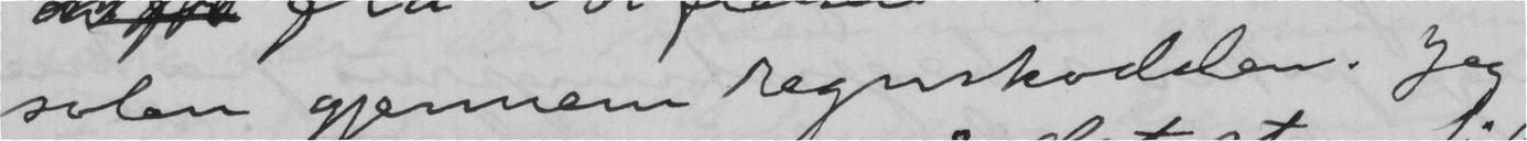solen gjennem regnskodden. Jeg
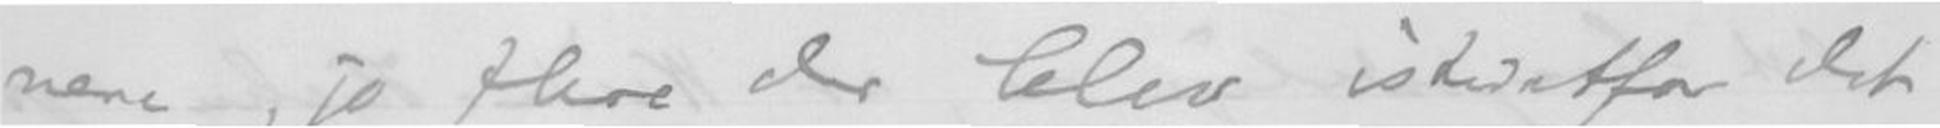nere, jo flere der blev istedfor det
